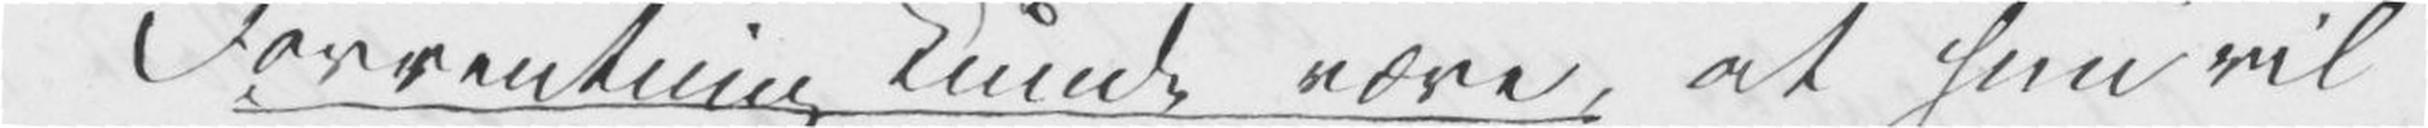Forrentning kunde være, at hun vil
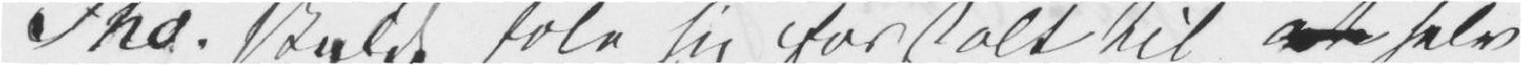Tho. skulde føle sig for stolt til at selv
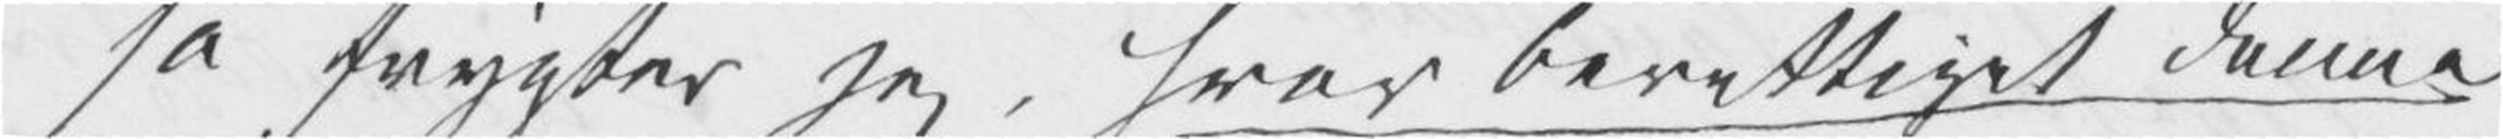så frygter jeg, hvor berettiget denne
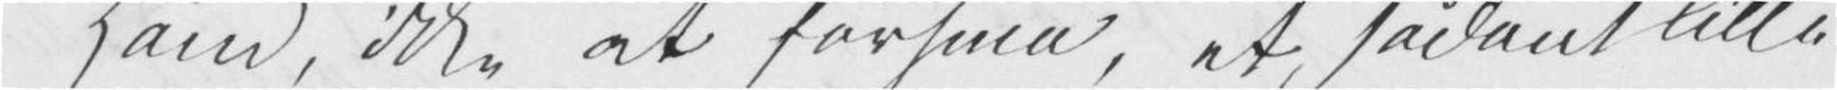Hånd, ikke at forsmå, et sådant lille
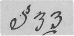§ 33
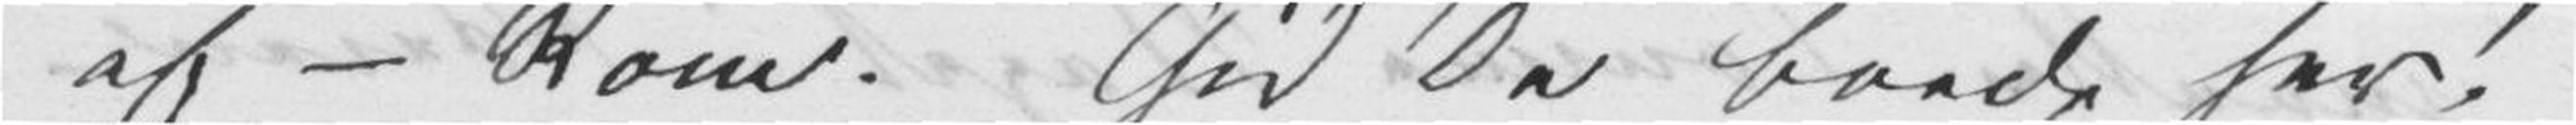af – Rom. Gid De boede her!
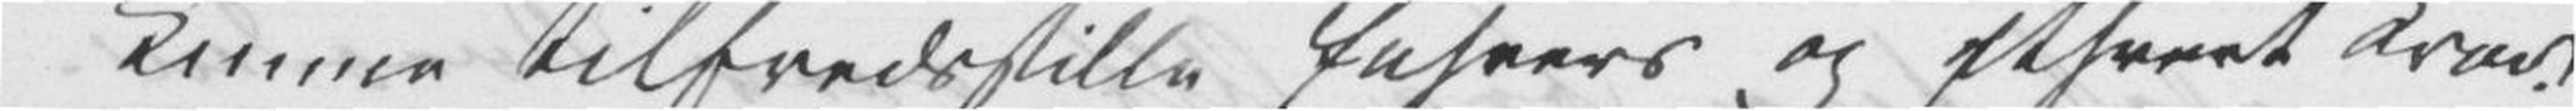kunne tilfredsstille Enhvers og Ethvert Krav.
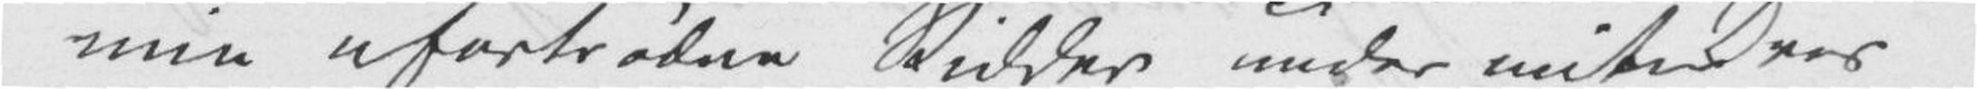min ufortrødne Ridder under mit Dres¬
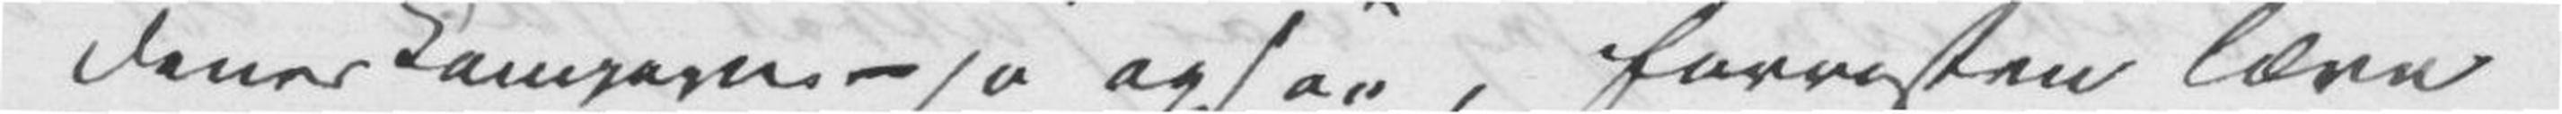dener kompagni – jo ogsaa, forresten lære
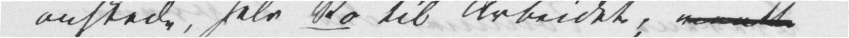ønskede, selv Ro til Arbeidet, maatte
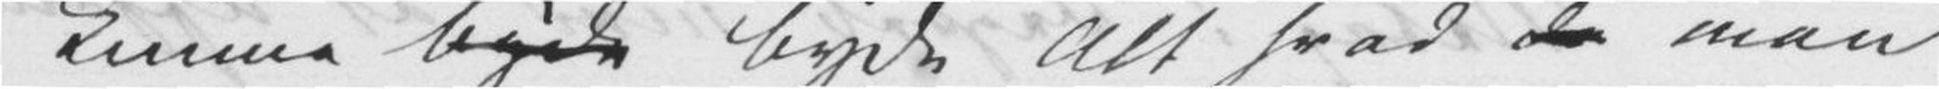kunne byde byde Alt hvad man
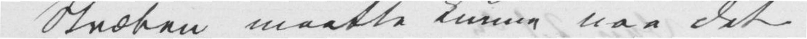Stræben maatte kunne naa det
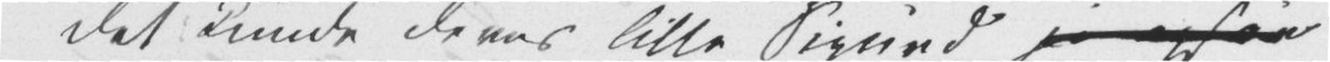Det kunde Deres lille Sigurd jo ogsaa
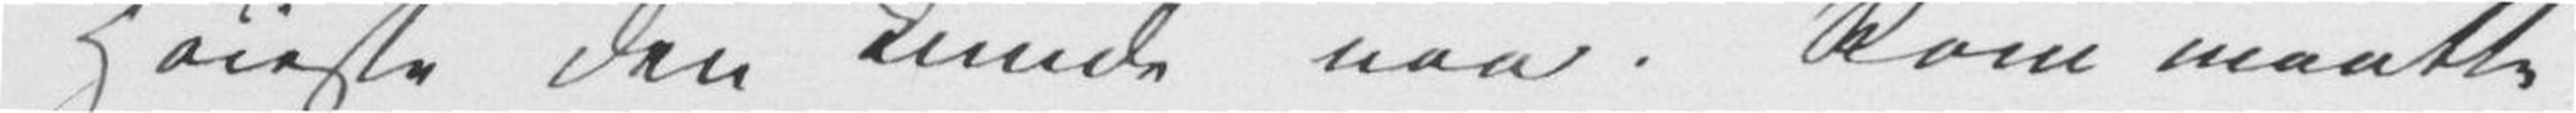høieste den kunde naa. Rom maatte
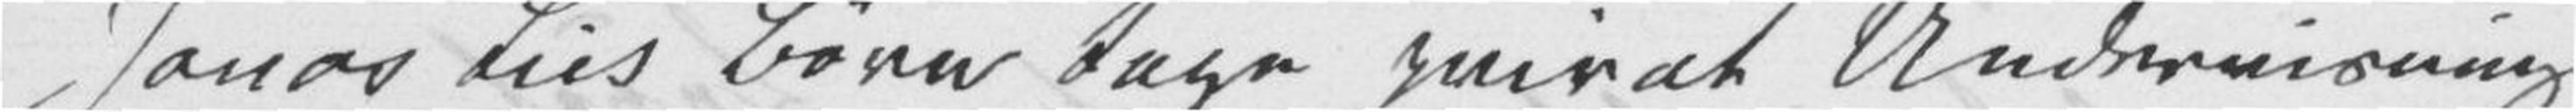Jonas Lies Børn tage privat Undervisning[.]
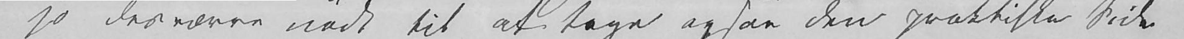jo desværre nødt til at tage ogsaa den praktiske Side
